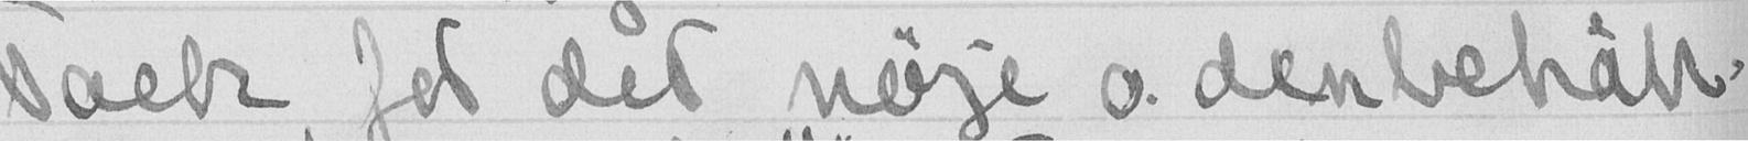tach for det nøje o. den behåll
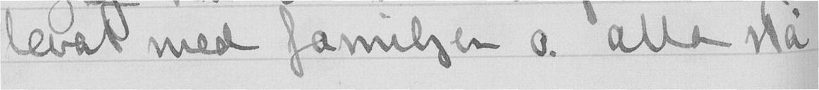levat med familjen o. alle nå
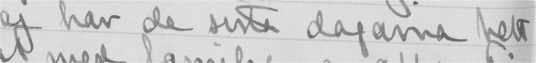Jag har de siste dagarna helt
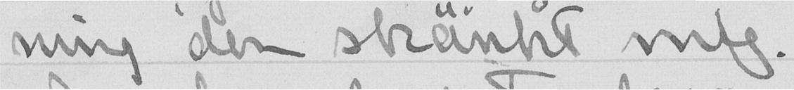mig den skönhet mig.
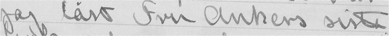jag lärt Fru Ankers siste
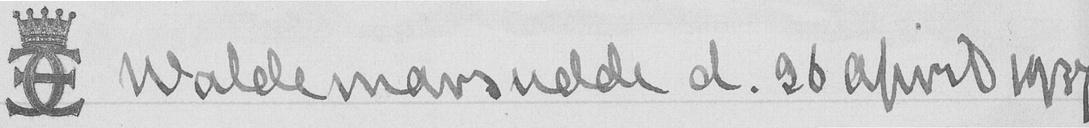Waldenarsvelde d. 26 april 1937
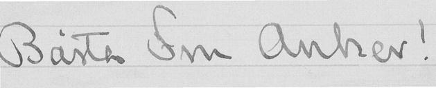Bäste Fru Anker!
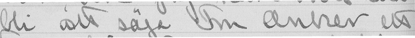bli at säga Fru Anker et
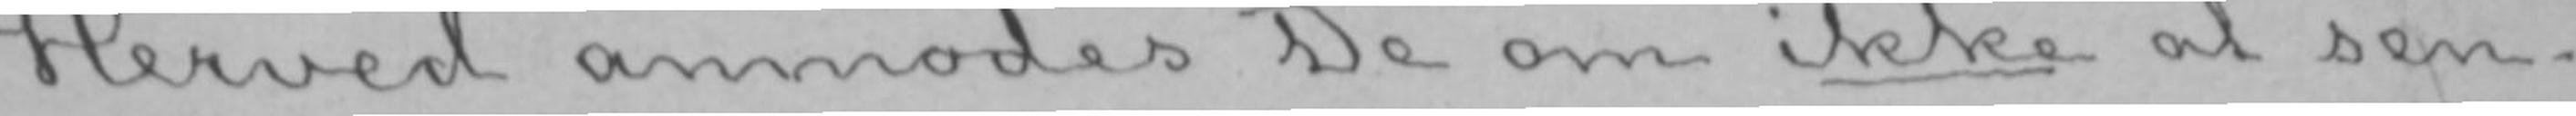Herved anmodes De om ikke at sen-
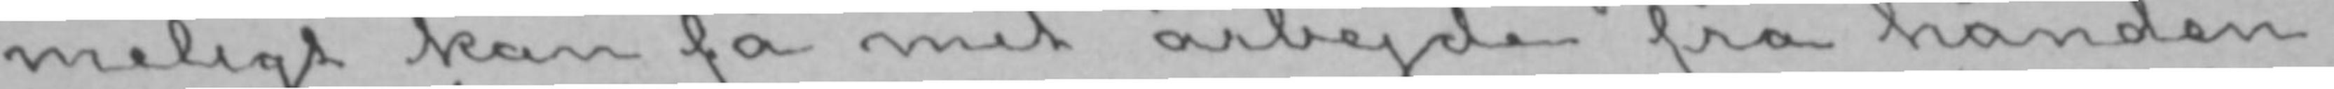meligt kan få mit arbejde fra hånden.
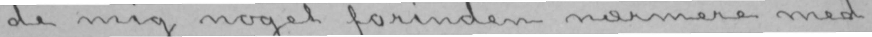de mig noget forinden nærmere med-
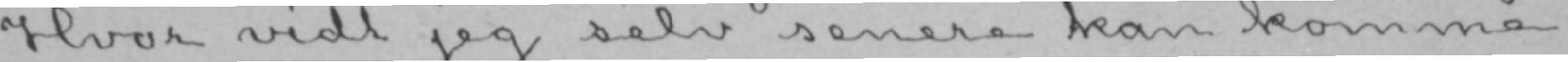Hvor vidt jeg selv senere kan komme
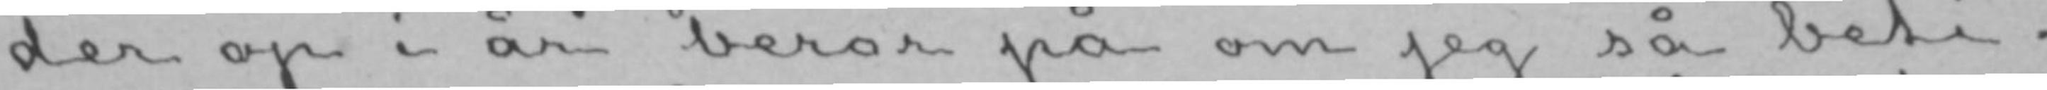der op i år beror på om jeg så beti-
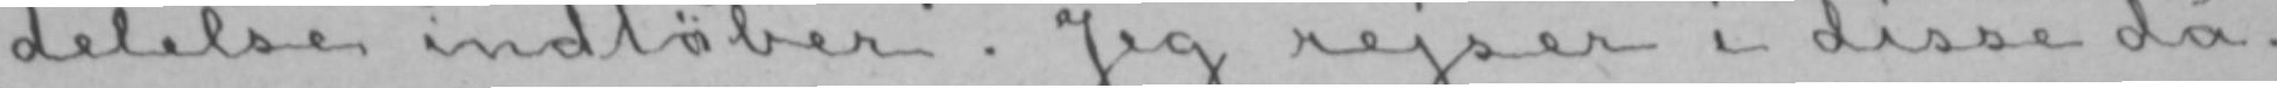delelse indløber. Jeg rejser i disse da-
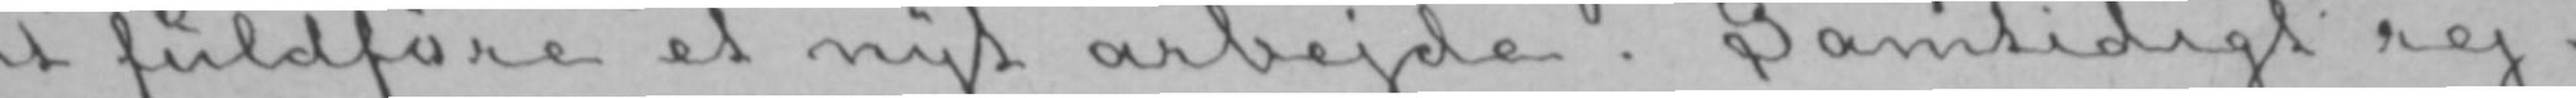at fuldføre et nyt arbejde. Samtidigt rej-
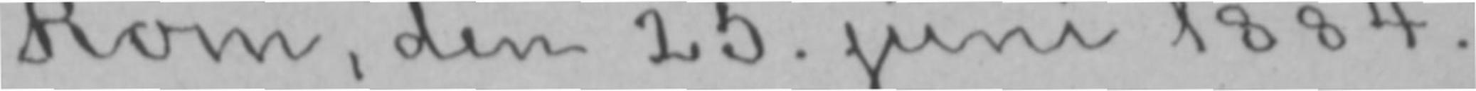Rom, den 25. juni 1884.
